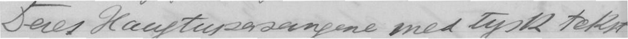Deres Haugtussasangene med tysk teskt
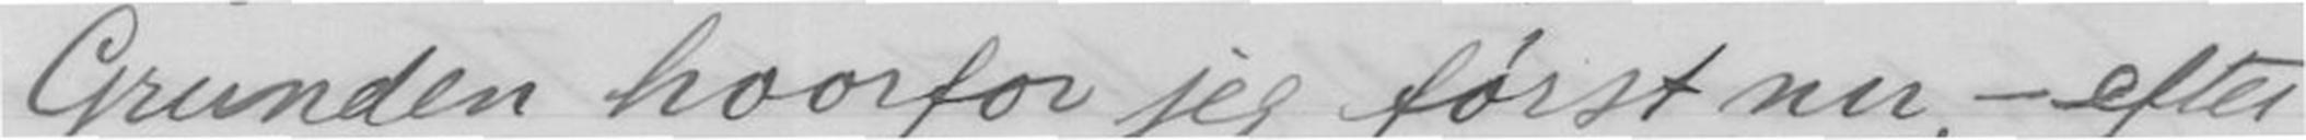Grunden hvorfor jeg først nu, - efter
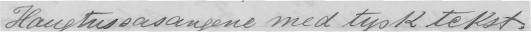Haugtussasangene med tysk tekst.
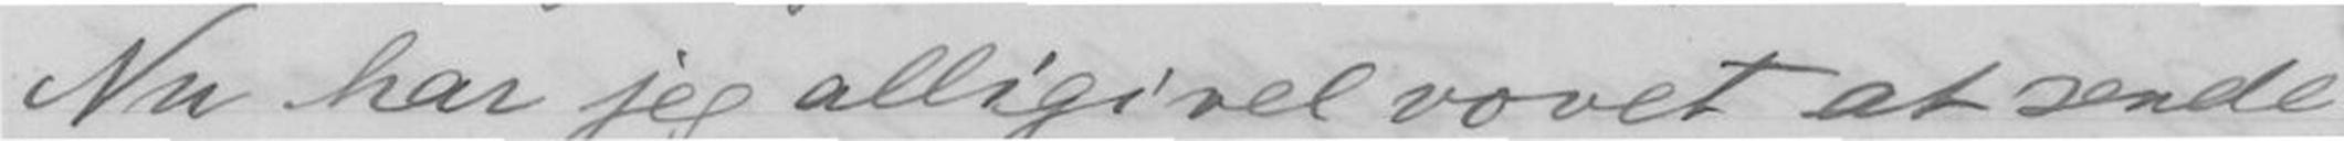Nu har jeg alligevel vovet at sende
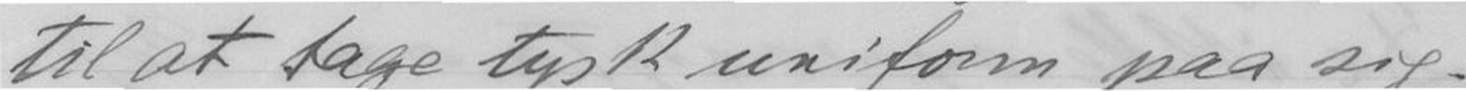til at tage tysk uniform paa sig.
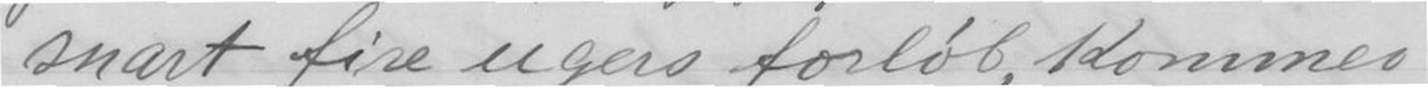snart fire ugers forløb, kommer
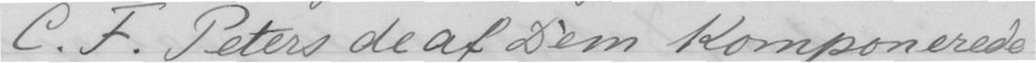C. F. Peters de af Dem Komponerede
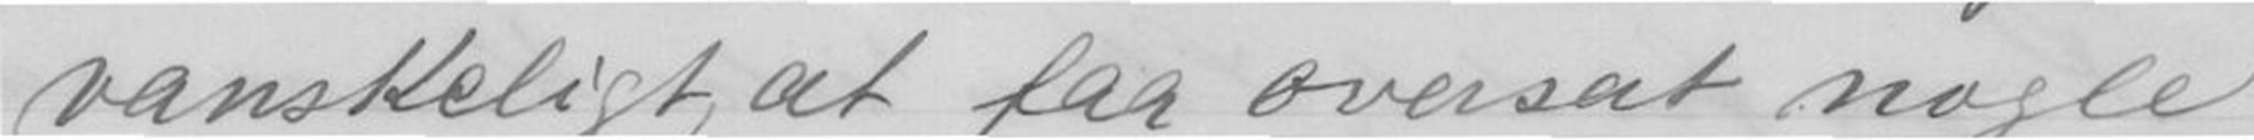vanskeligt, at faa oversat nogle
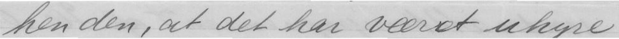den, at det har været uhyre
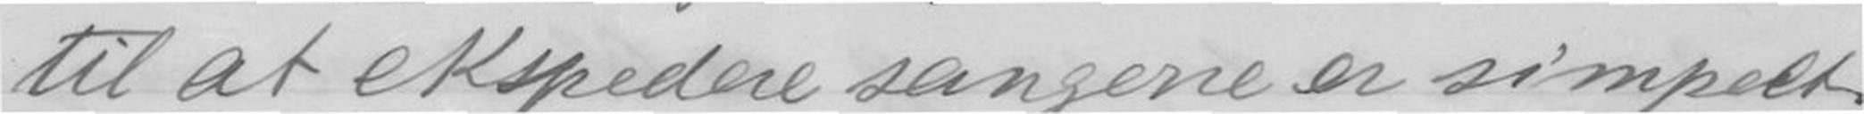til at ekspedere sangene er simpelthen
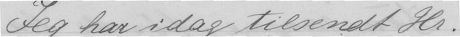Jeg har idag tilsendt Hr.
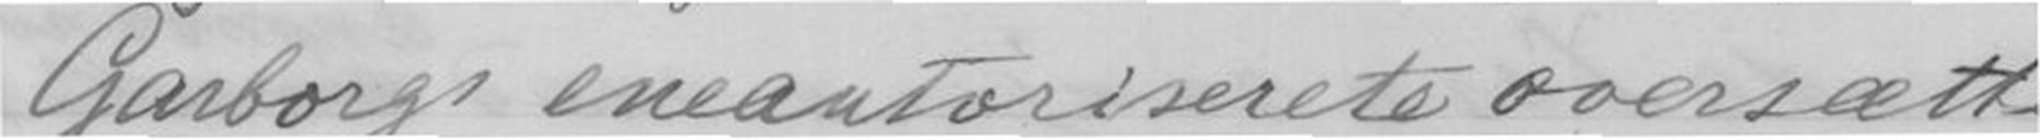Garborgs eneautoriserete oversætt
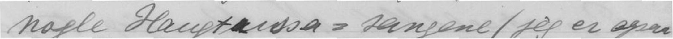nogle Haugtussa=sangene (jeg er ogsaa
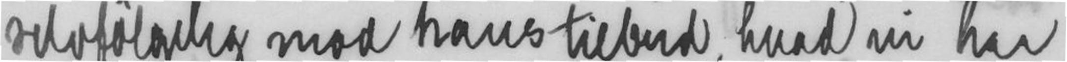selvfølgelig mod hans tilbud, hvad vi har
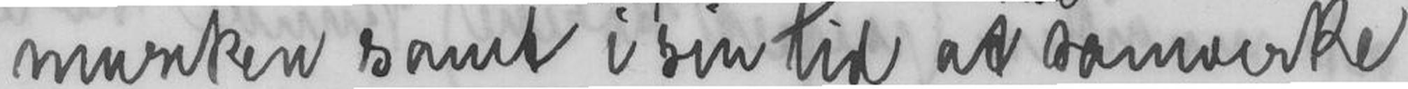musiken samt hans sin tid at samvirket
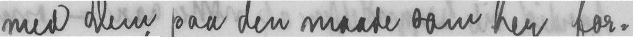med Dem, paa den maade som her for-
